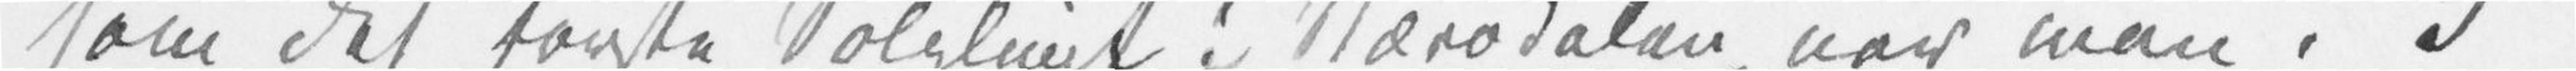som det første Solglimt i Nærødalen, når man i 3
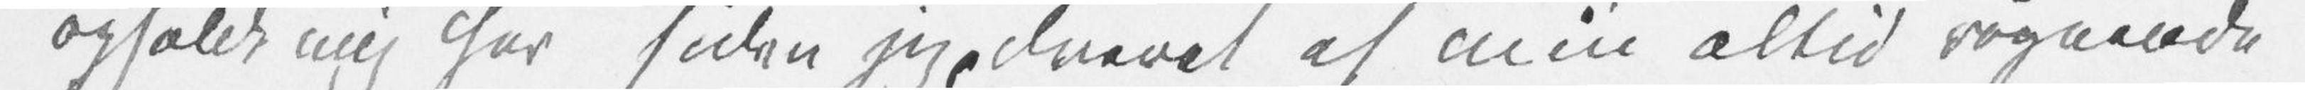opholdt mig her siden jeg, drevet af min altid vågnende
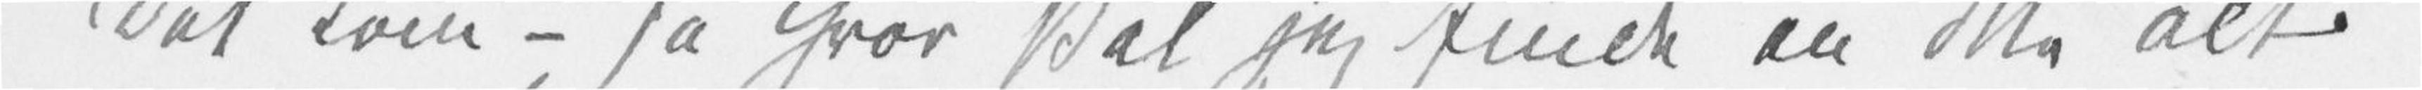Det kom – ja hvor skal jeg finde en ikke alt
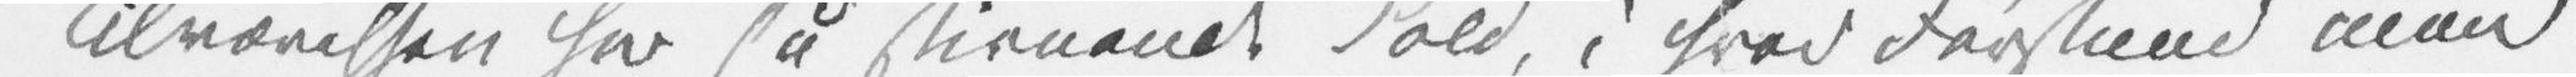Tilværelsen her så stivnende kold, i hvad Forstand man
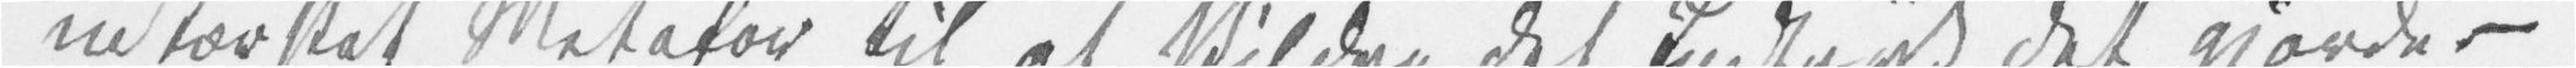udtærsket Metafor til at skildre det Indtryk det gjorde? –
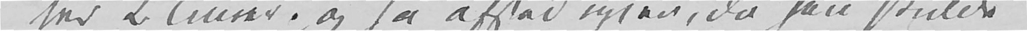her 2 Timer, og så afsted igjen, da han skulde
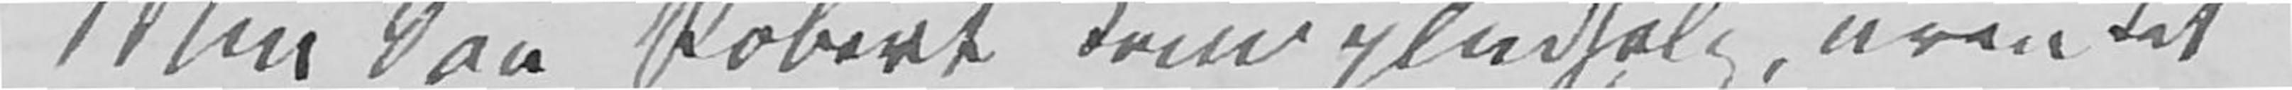Min Søn Robert kom pludselig, uventet
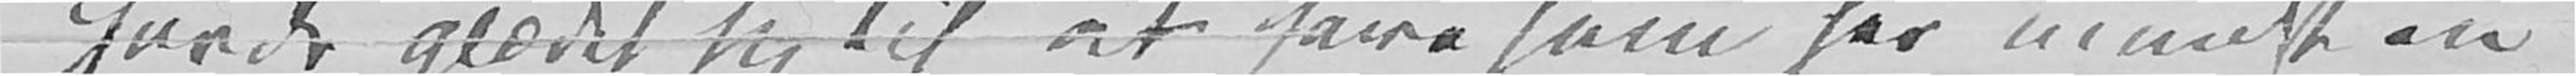havde glædet sig til at have ham her mindst en
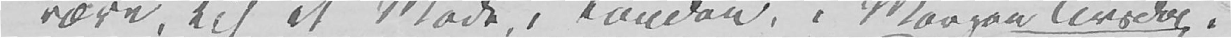være, til et Møde, i London, i Morgen Tirsdag.
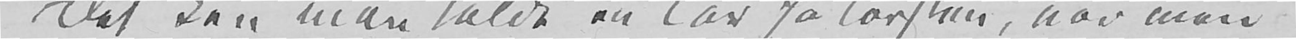Det kan man kalde en Tår på Tørsten, når man
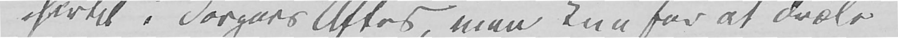hertil i Forgårs Aftes, men kun for at dvæle
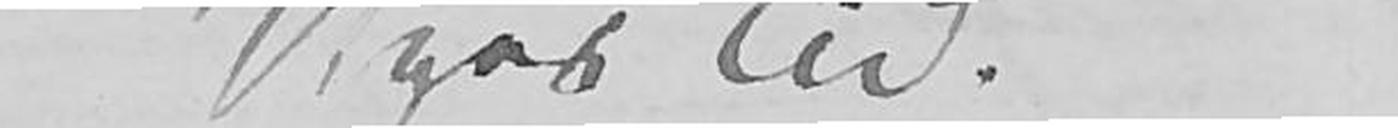Uges Tid.
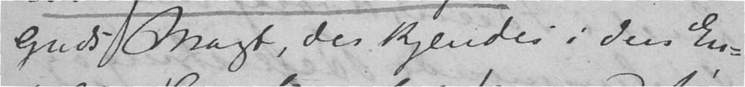Guds Magt, der kjendes i den En-
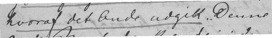hvoraf det Onde udgik. Denne
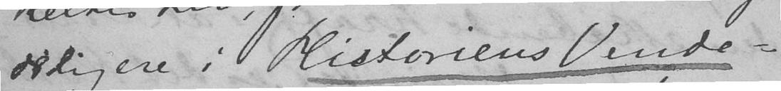deligere i Historiens Vende-
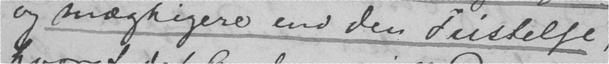og mægtigere end den Fristelse,
